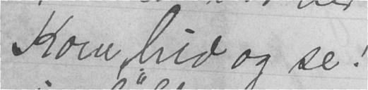Kom hid og se!
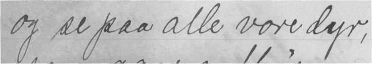og se paa alle vore dyr,
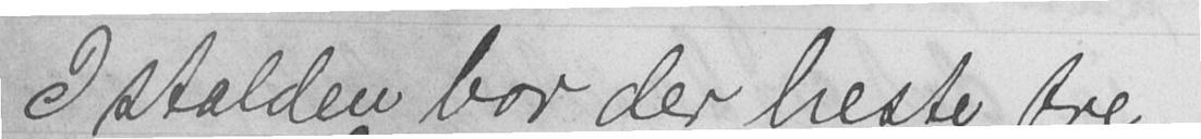I stalden bor der heste tre.
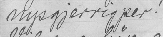nysgjerrigper!
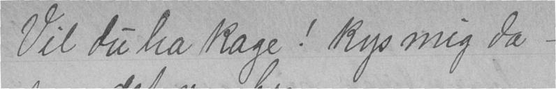Vil du ha kage! kys mig da -
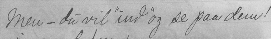Men - du vil "ind" og se paa dem!
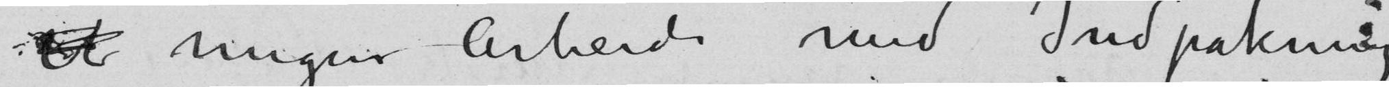et megen Arbeide med Indpakning
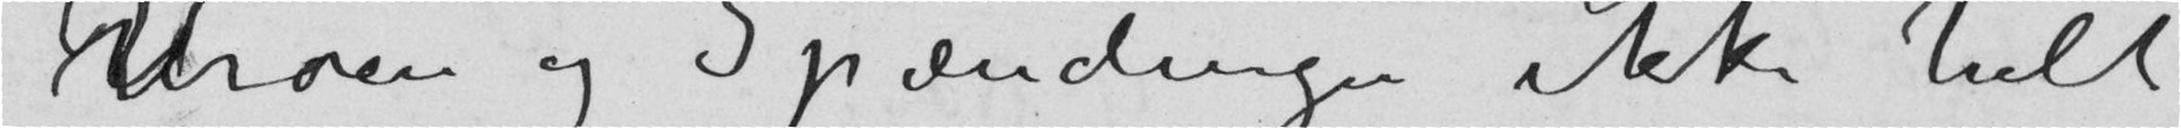Uroen og Spændingen ikke helt
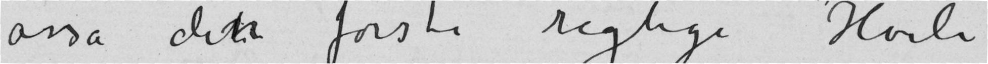osså detn første rigtige Hvile
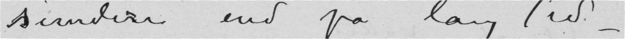sundere end på lang Tid –
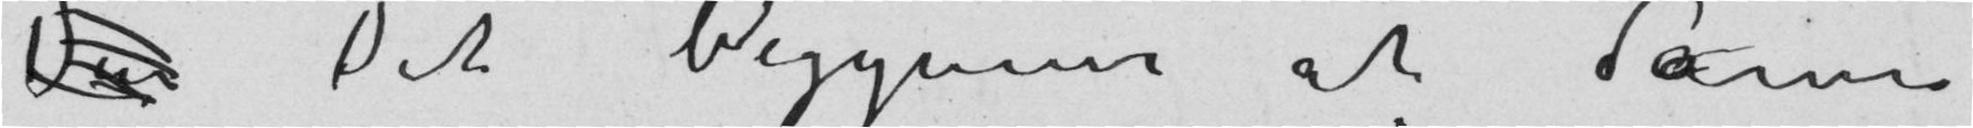D Det begynner at danne
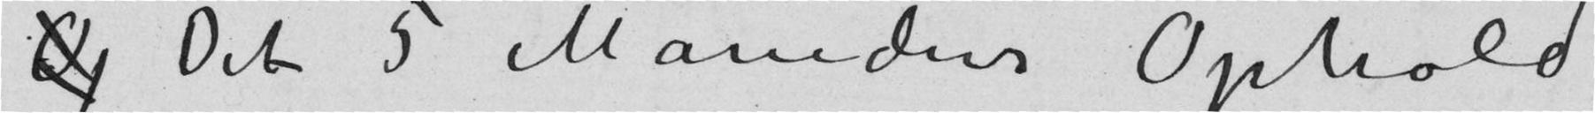Og Det 5 Måneders Ophold
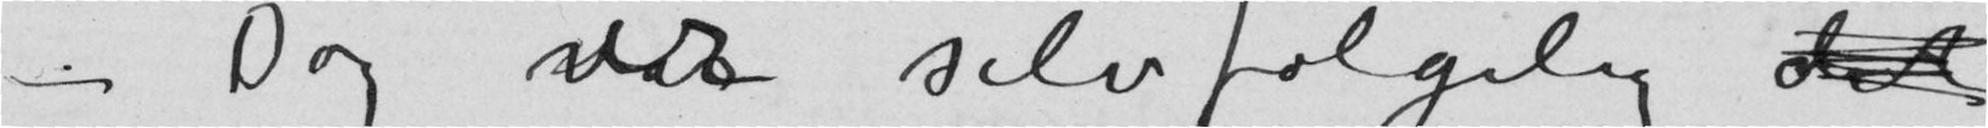– Dog varer selvfølgelig det
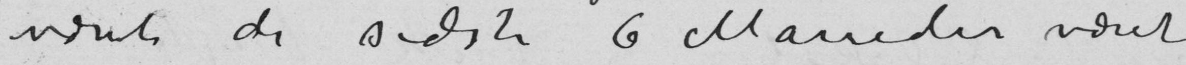været de sidste 6 Måneder været
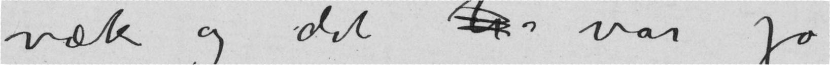væk og det har var jo
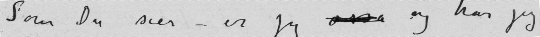Som du sier – er jeg osså og har jeg
